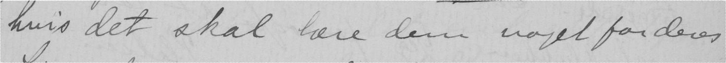hvis det skal lære dem noget for deres
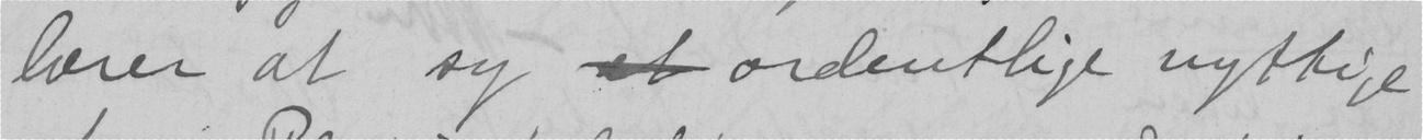lærer at sy et ordentlige nyttige
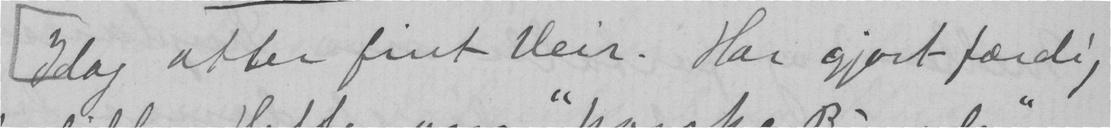Idag atter fint veir. Har gjort færdig
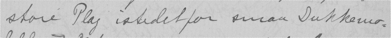store Plag istedetfor smaa Dukkemo-
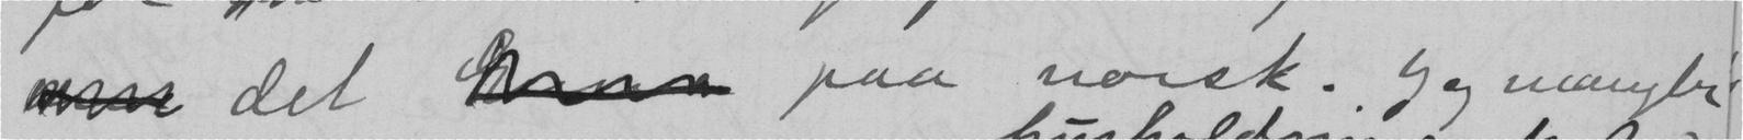det  paa norsk. Jeg mangler
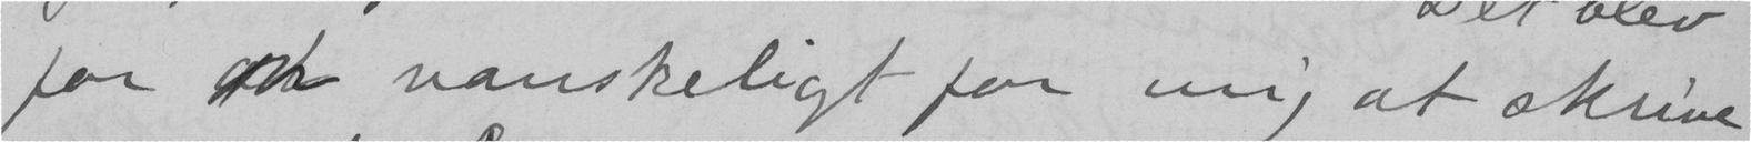for  vanskeligt for mig at skrive
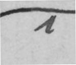i
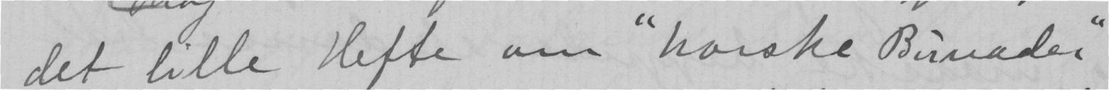det lille Hefte om "norske Bunader"
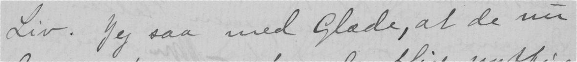Liv. Jeg saa med Glæde, at de nu
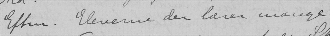efterm. Eleverne der lærer mange
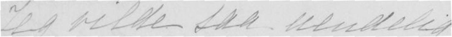Jeg vilde saa uendelig
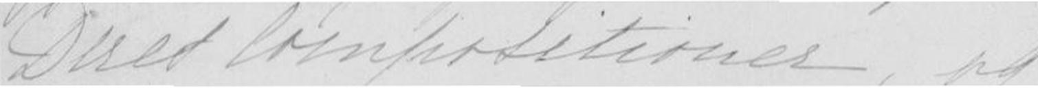Deres Compositioner, og
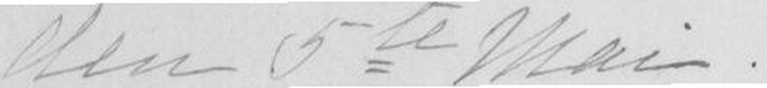den 5te Mai.
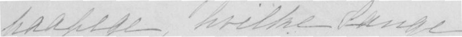paapege, hvilken Sange
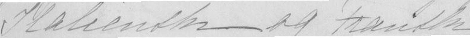Italienske og Franske,
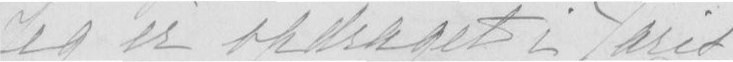Jeg er opdraget i Paris,
